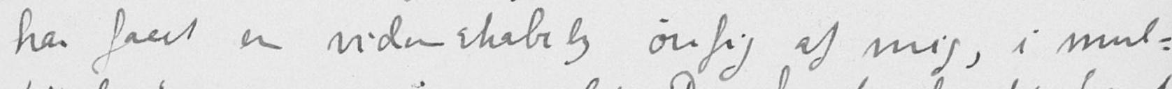har faaet en videnskabelig ørefig af mig, i mul-
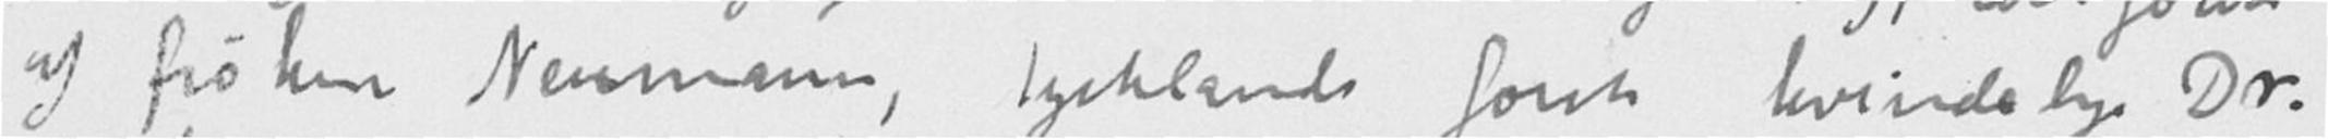af frøken Nessmann, Tysklands første kvindelige Dr.
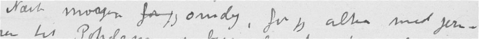Næste morgen, for jeg ondag, for jeg altsaa med jern-
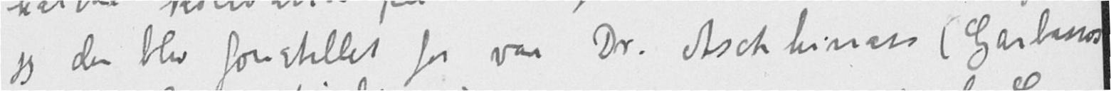jeg der blev forestillet for var Dr. Aschkinass (Garbassos
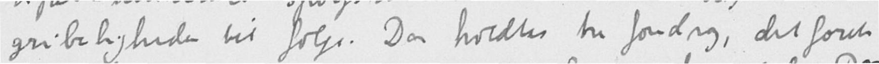gribeligheder til følge. Der holdtes tre foredrag, det første
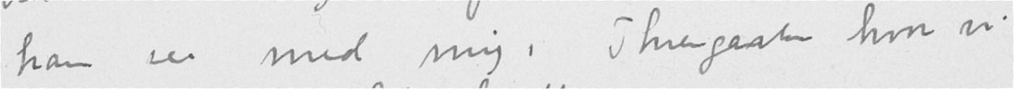ham saa med mig i Thiergarten hvor vi
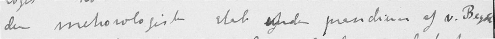den meteorologiske stab under prandium af v. Bezold
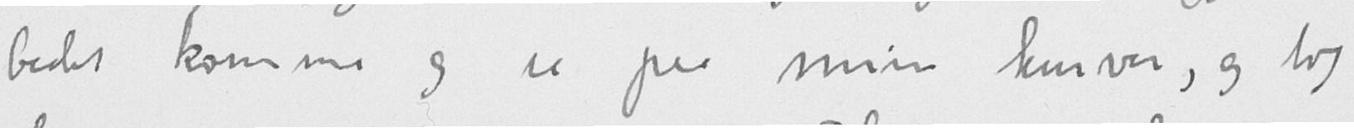bedet komme og se paa mine kurver, og tog
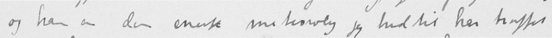og han er den eneste meteorolog jeg hidtil har truffet
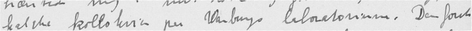kalske kollokvier paa Warburgs laboratorium. Den første
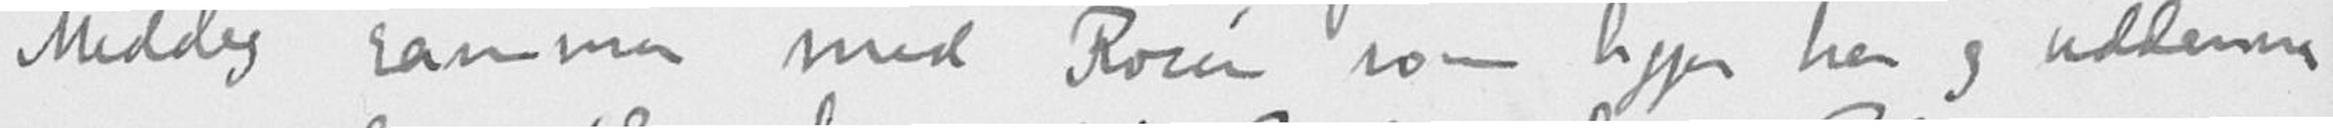Middag sammen med Rosén som ligger her og uddenne
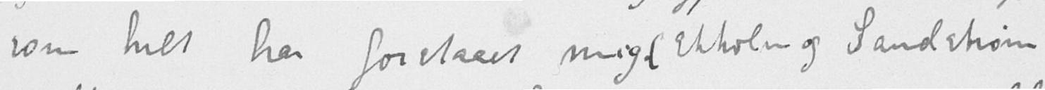som helt har forstaaet mig (Ekholm og Sandstrøm
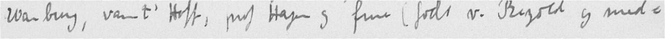Warburg, vant Hoff, prof Hagen og frue (født v. Bezold og med-
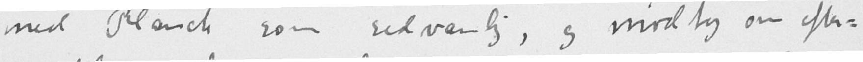med Planck som sedvanlig, og modtog om efter-
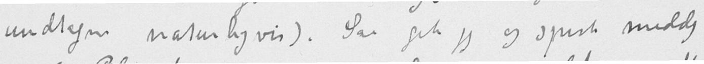undtagne naturligvis). Saa gik jeg og spiste middag
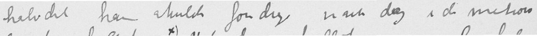halvdel han skulde foredrage næste dag i de meteoro-
